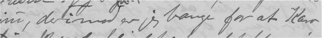nu, derimod er jeg bange for at Karo-
